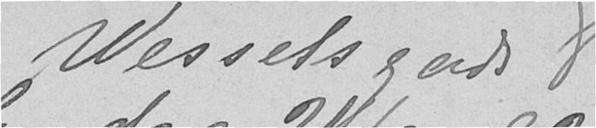Wessels gade 8
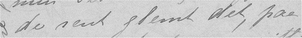de rent glemt det, paa
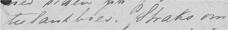tulantbrev. Straks om
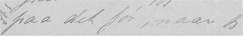paa det før, naar jeg
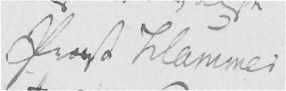Provst Hammer
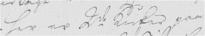her er 2de Kirker paa
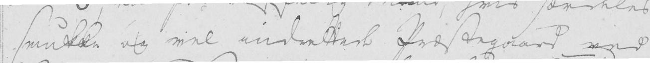smukke og vel indrettede Præstegaard ved
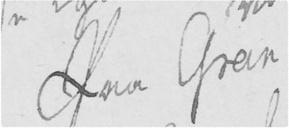Paa Gran
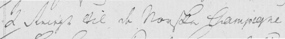2 Recept til de Norske Champagne
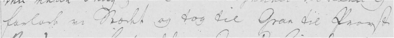forlod vi Stædet og tog til Gran til Provst
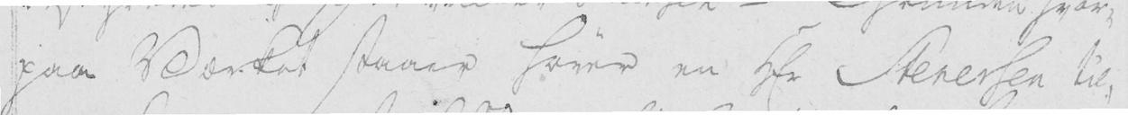paa Værket staaer hører en Hr Stenersen til,
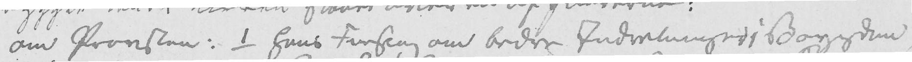om Provsten: 1 Hans Forslag om bedre Indretninger i Bøygden
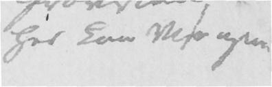her ham Vise igen
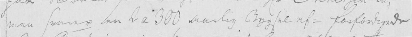men svarer en 2 a 300 aarlig Bygsel af - forfærdigede
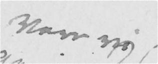vare vi
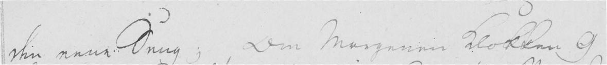den eene seng; Om Morgenen Klokken 9
